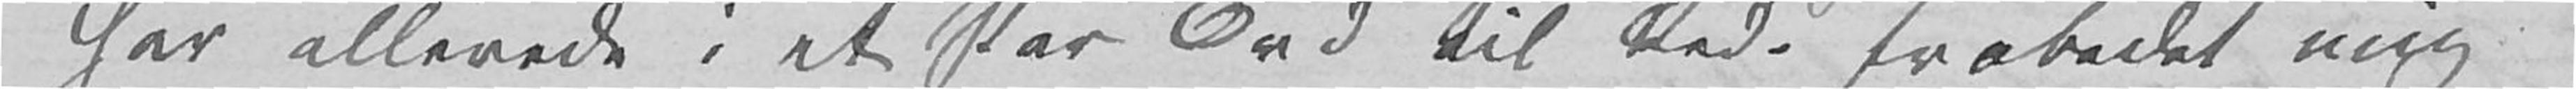har allerede i et Par Ord til Red. frabedet mig
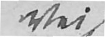Vei
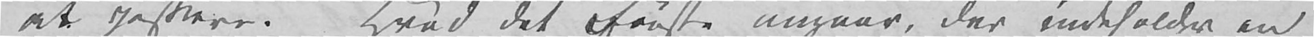at passere. Hvad det første angaar, der indeholder en
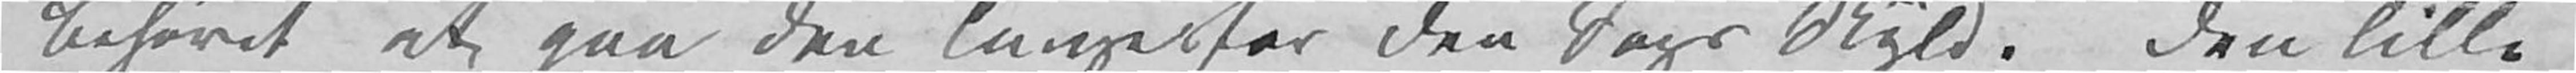behøvet at gaa den lange for den Sags Skyld. Den lille
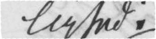lighed.
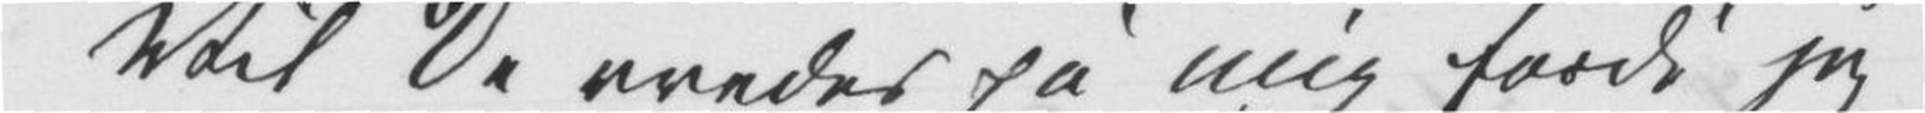Vil De vredes på mig fordi jeg
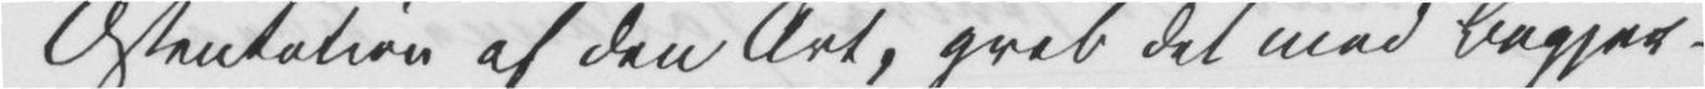Ostentation af den Art, greb det med Begjer¬
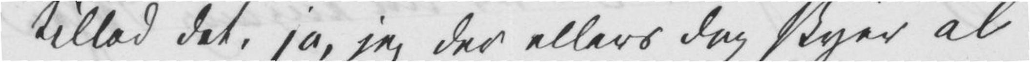tillod det, ja, jeg der ellers dog skyer al
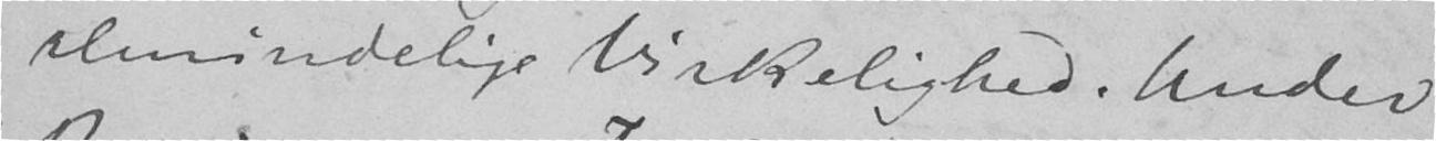almindelige Virkelighed. Under
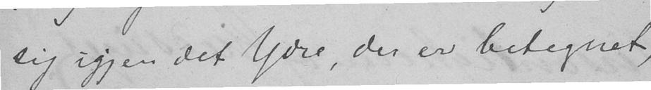sig igjen det ydre, der er betegnet,
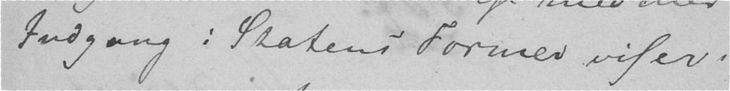Indgang i Statens Former viser
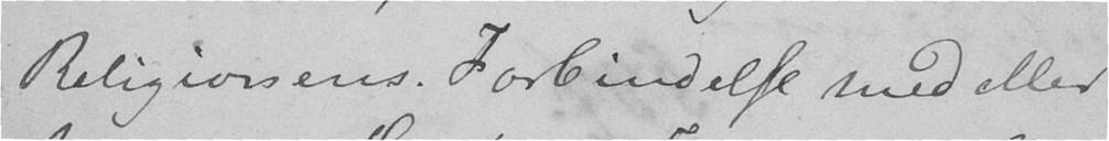Religionens Forbindelse med eller
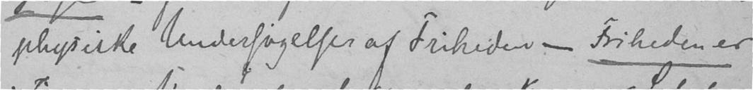physiske Undersøgelser af Friheden – Friheden er
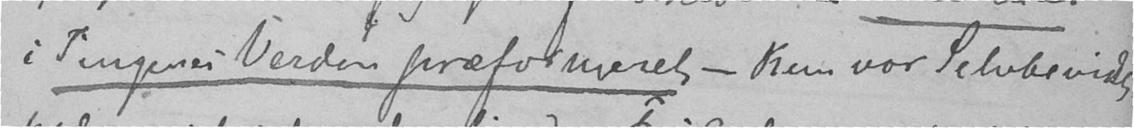i Tingenes Verden præformeret – kun vor Selvbevidst-
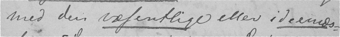med den væsentlige eller ideemæs-
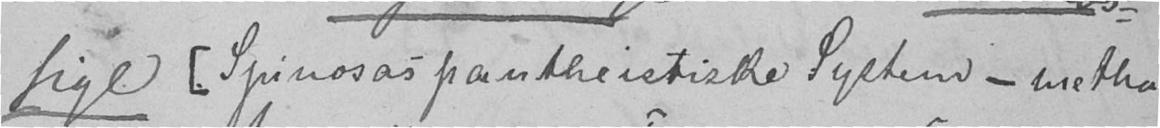sige [Spinozas pantheitiske System – metha-
